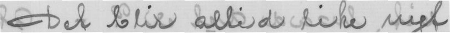Det blir altid like nyt
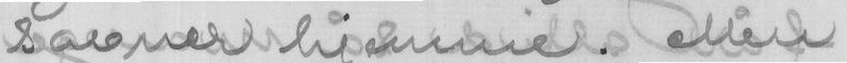savner hjemme. Men
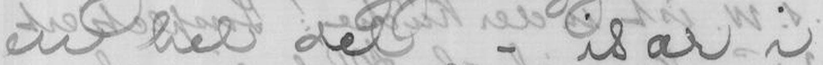en hel del, - især i
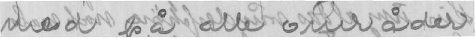med på alle områder.
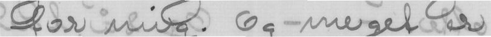for mig. Og meget er
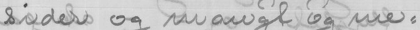sider og mangt og me-
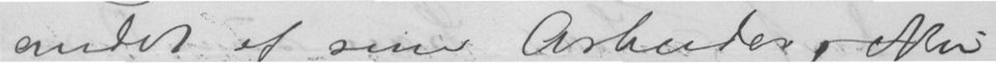andet af sine Arbeider, Nu
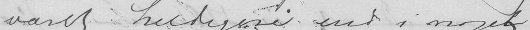været heldigere end i noget
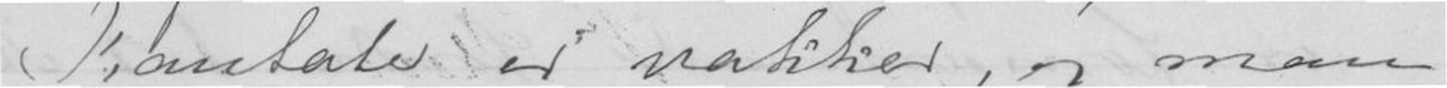Kantate er vakker, og man
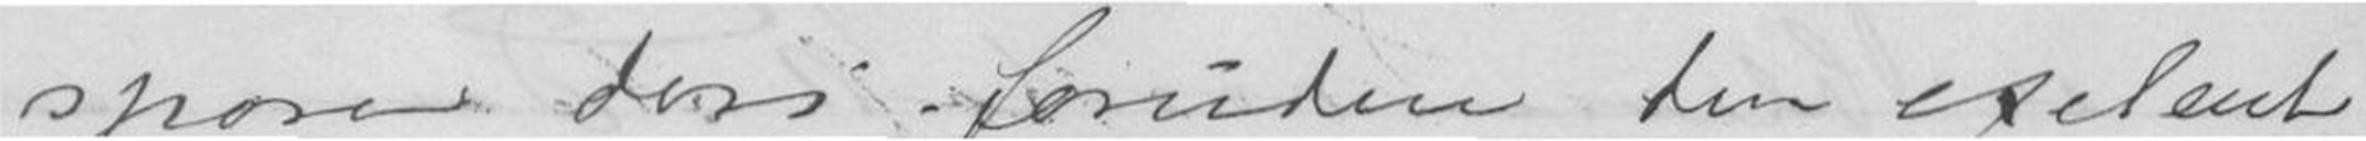sporer deri foruden den exelent
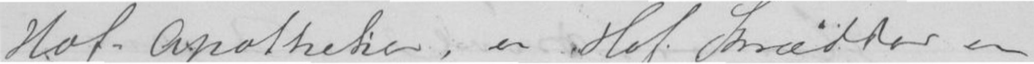Hof. Apotheker, en Hof Skrædder en
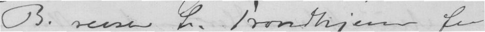B. reise til Trondhjem for
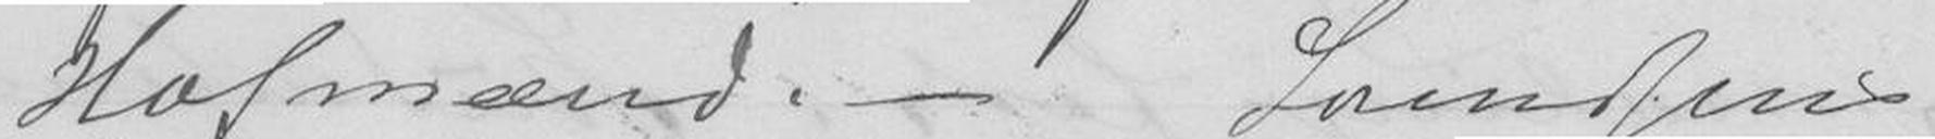Hofmænd. - Svendsens
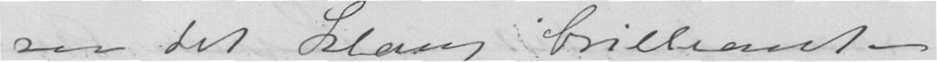om det Klang brilliant -
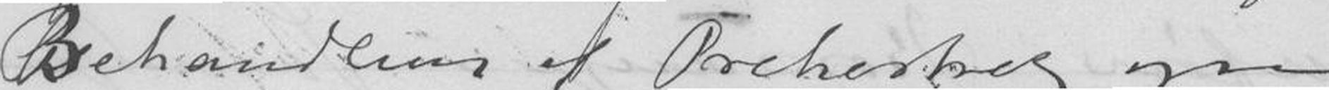Behandling af Orchestret ogsaa
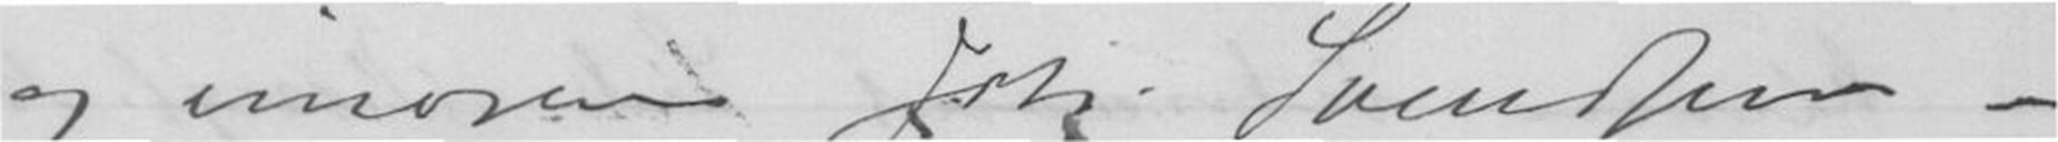og imorgen Joh. Svendsen -
